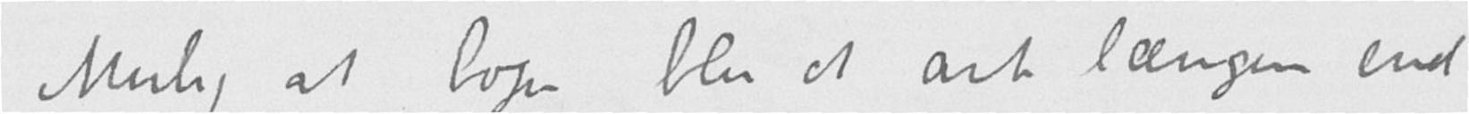Mulig at bogen blir et ark længere end
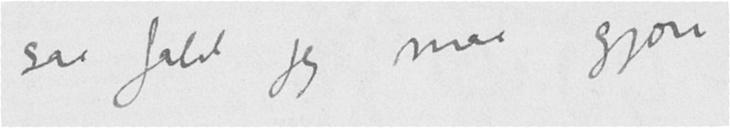saa fald jeg maa gjøre
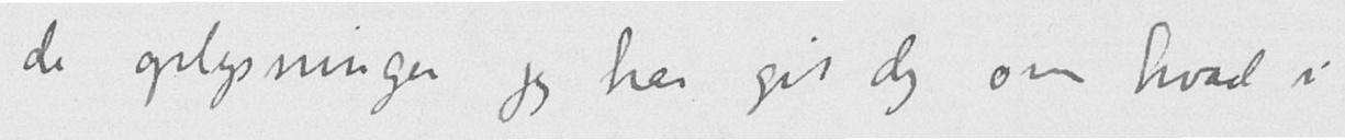de oplysninger jeg har git dig om hvad i
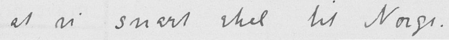at vi snart skal til Norge.
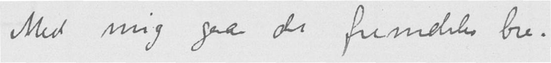Med mig gaar det fremdeles bra.
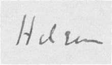Hilsen
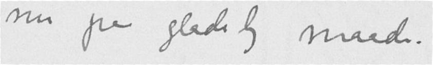nu paa glædelig maade.
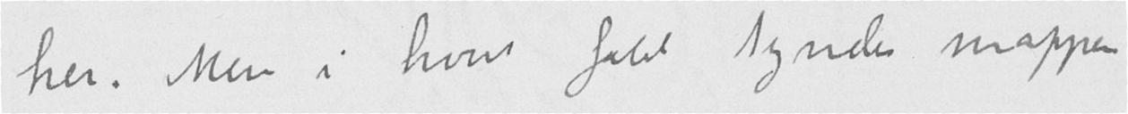her. Men i hvert fald tyndes mappen
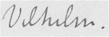Vilhelm.
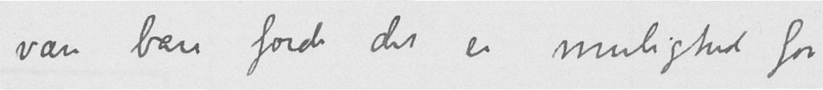være bare fordi det er mulighed for
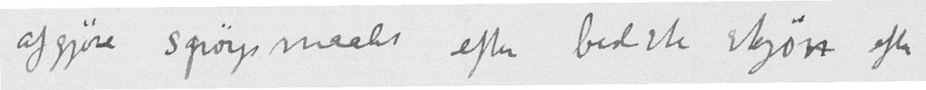afgjøre spørgsmaalet efter bedste skjøn efter
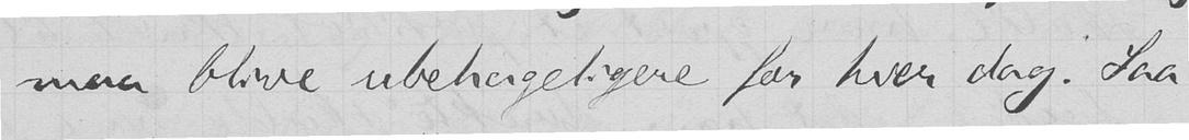maa blive ubehageligere for hver dag. Saa
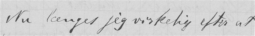Nu længes jeg virkelig efter at
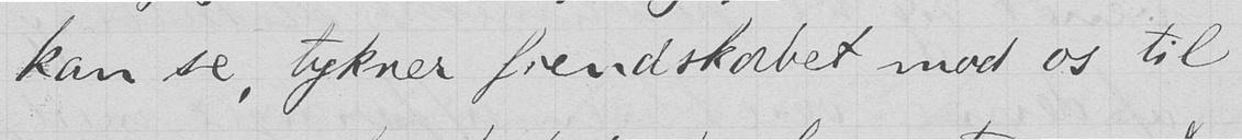kan se, tykner fiendskabet mod os til
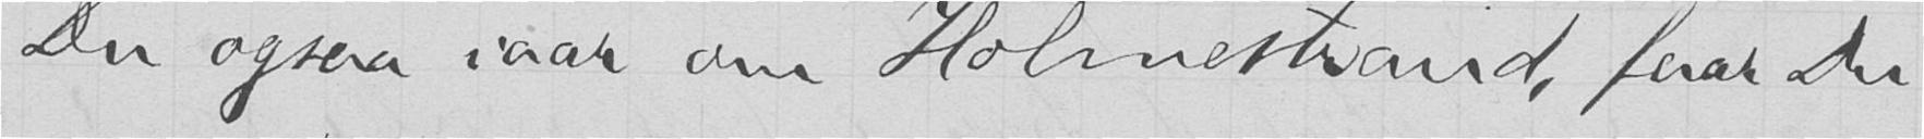Du ogsaa iaar om Holmestrand, faar Du
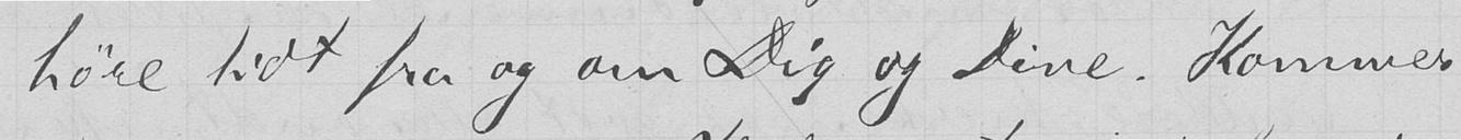høre lidt fra og om Dig og Dine. Kommer
